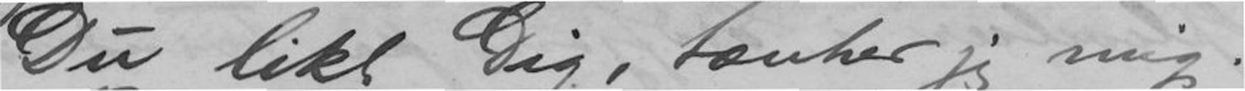Du likt Dig, tænker jeg mig.
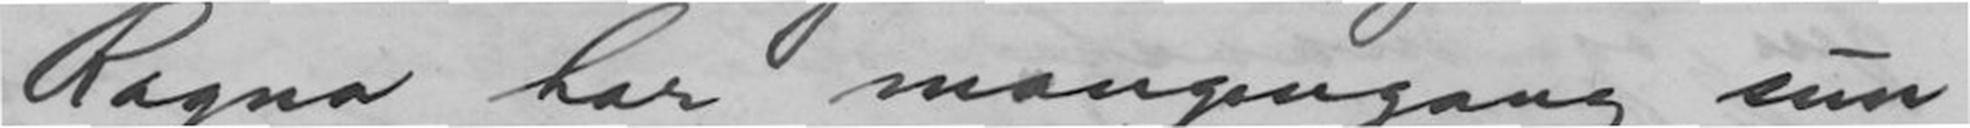Ragna har mangengang sun-
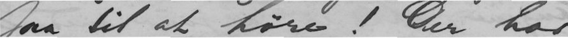saa til at høre! Der har
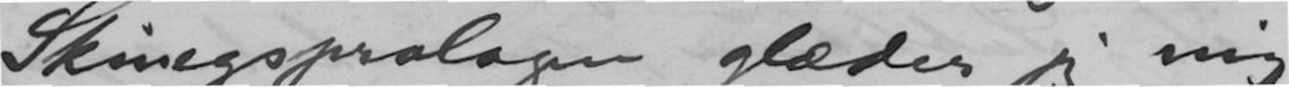Skinegsprologen glæder jeg mig
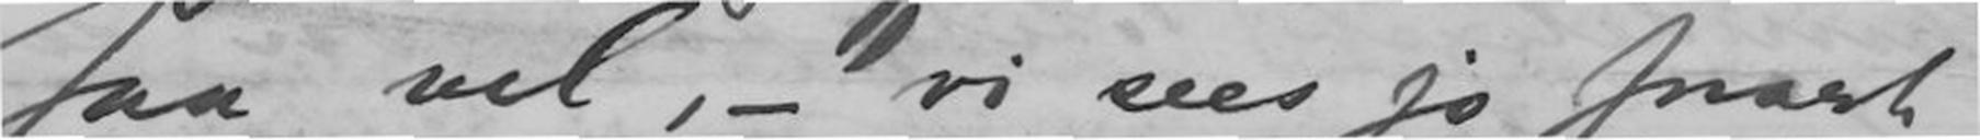saa vel, - "vi sees jo snart"!
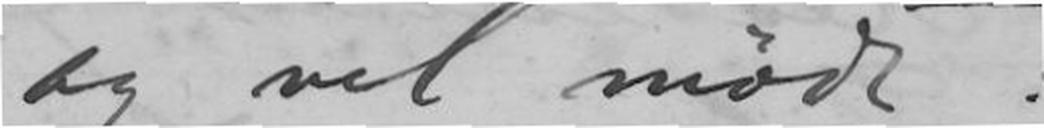og vel mødt!
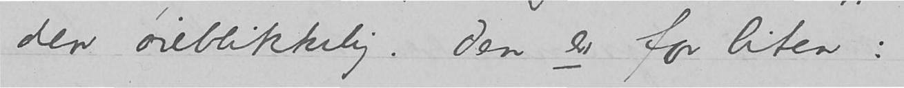den øieblikkelig. Den er for liten:
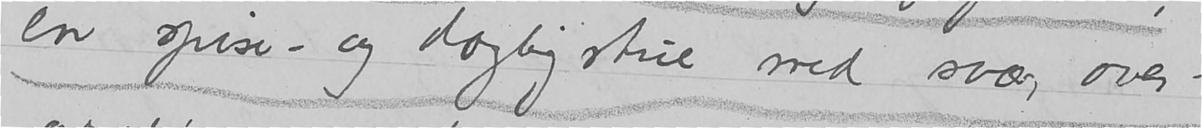en spise- og dagligstue med svær over-
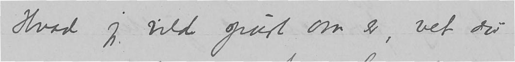Hvad jeg, vilde spurt om er, vet du
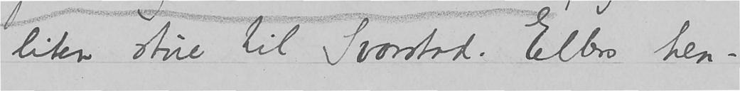liten stue til Svarstad. Ellers hen-
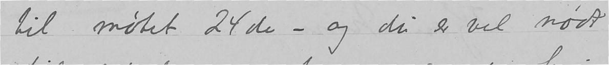til møtet 24de – og du er vel nødt,
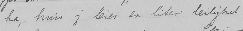ha, hvis jeg leier en liten leilighet
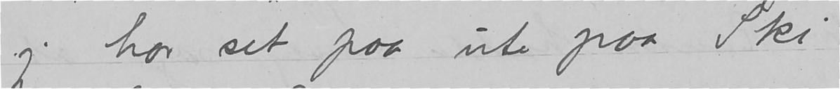jeg har set paa ute paa Ski –
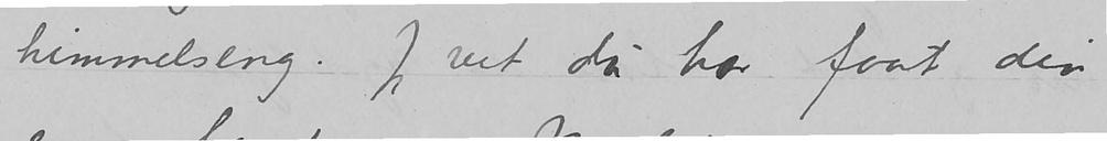himmelseng. Jeg vet du har faat din
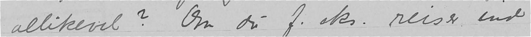allikevel? Om du f. eks. reiser end
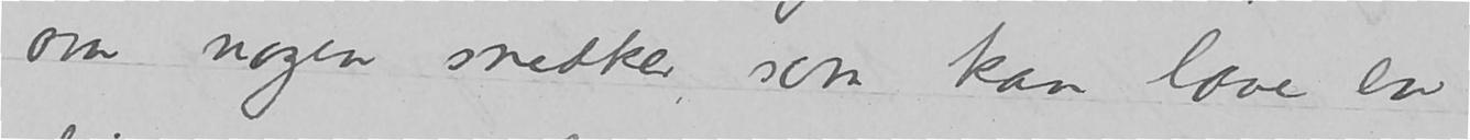om nogen snedker, som kan lave en
